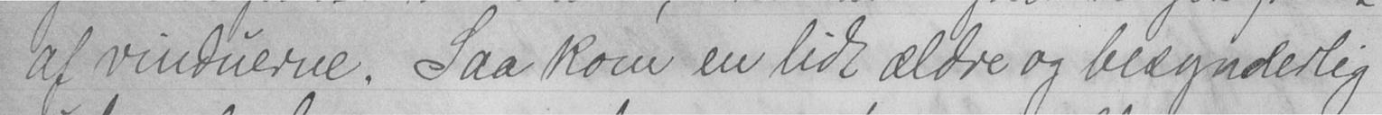af vinduerne. Saa kom en lidt ældre og besynderlig
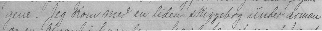gene. Jeg kom med en liden skizzebog under armen
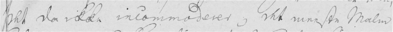det da ikke incommoderer; det meeste Malm
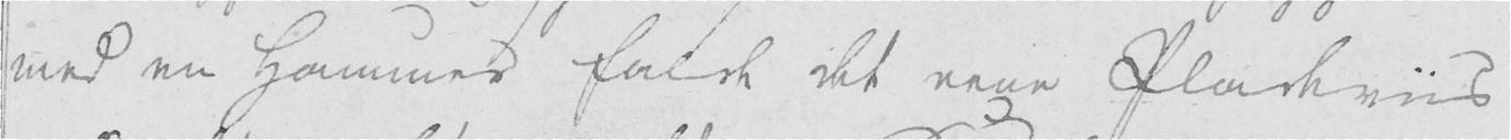med en Hammer falde det eene Pladeviis
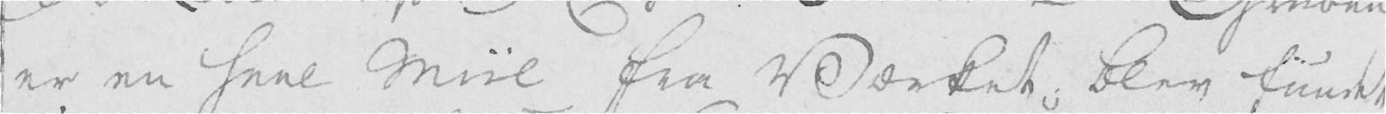er en heel Miil fra Værket; blev fundet
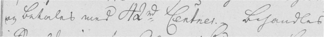og betales med 42 rd Centner. behandles
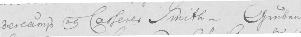edercamp og Casserer Smith - Gruben
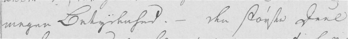megen Betydenhed. - Den største Deel
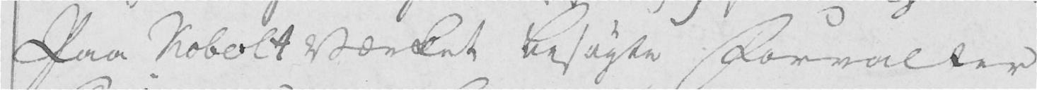Paa Kobolt Værket besøgte Forvalter
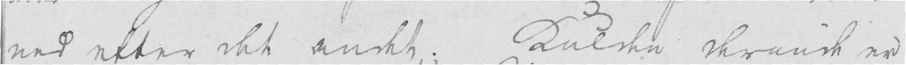ned efter det andet; Kulden derinde er
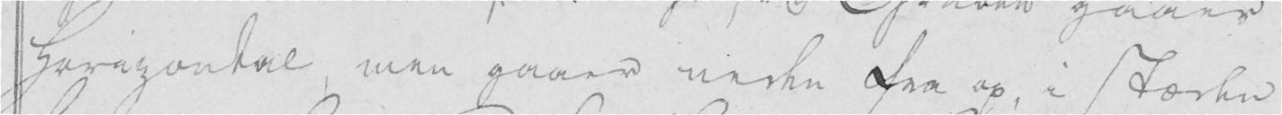horizontal, men gaaer neden fra op, i stæden
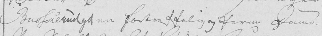Busscerud gd en fortreffelig og ferm Dame. -
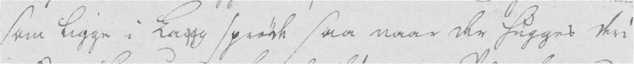som ligge i Lag sprøde saa naar der hugges deri
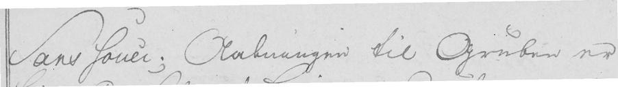Sans souci; Aabningen til Gruben er
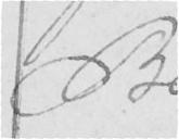B
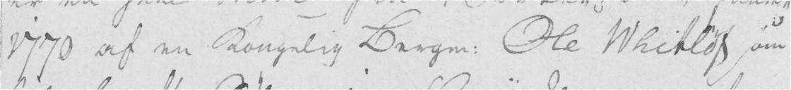1770 af en Kongelig Bergm. Ole Whitløf som
